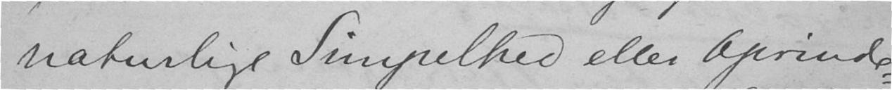naturlige Simpelhed eller Oprinde-
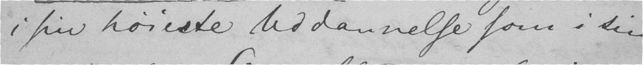i sin høieste Uddannelse som i sin
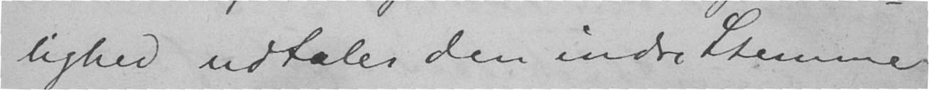lighed udtaler den indre Stemme
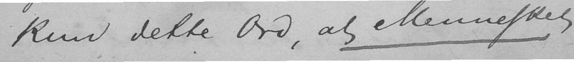kun dette Ord, at Mennesket
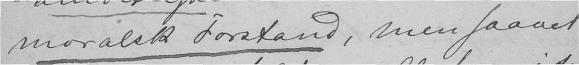moralsk Forstand, men saavel
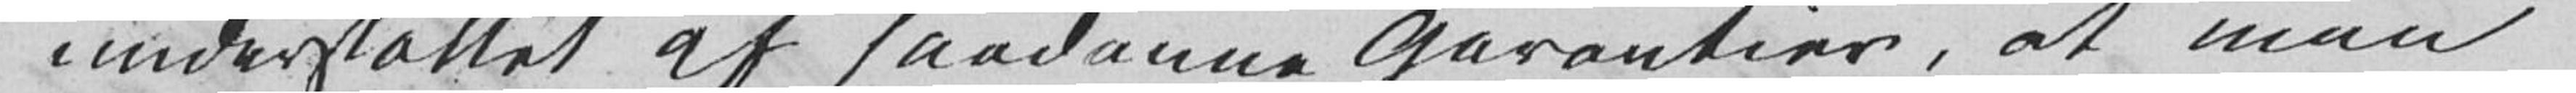understøttet af saadanne Garantier, at man
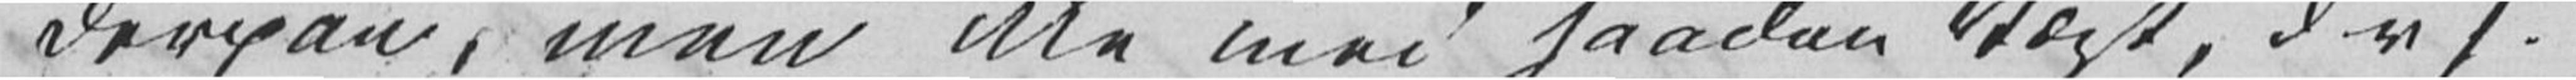derpaa, men ikke med saadan Vægt, d v s.
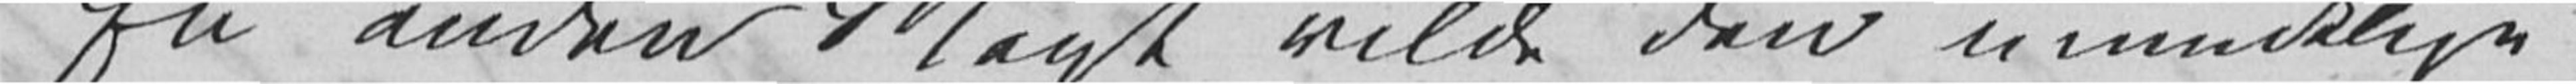En anden Magt vilde den mundtlige
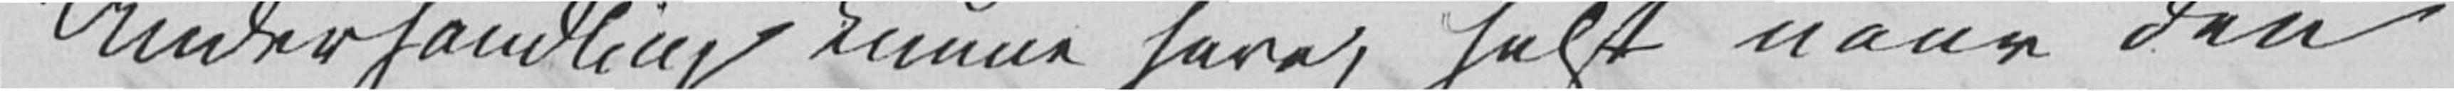Underhandling kunne have, helst naar den
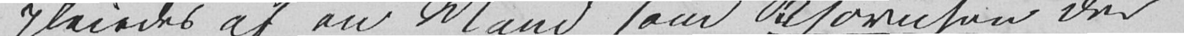pleiedes af en Mand som Bjørnson der
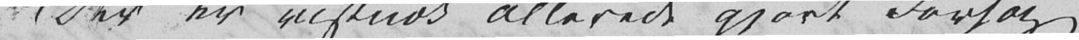Der er vistnok allerede gjort Forsøg
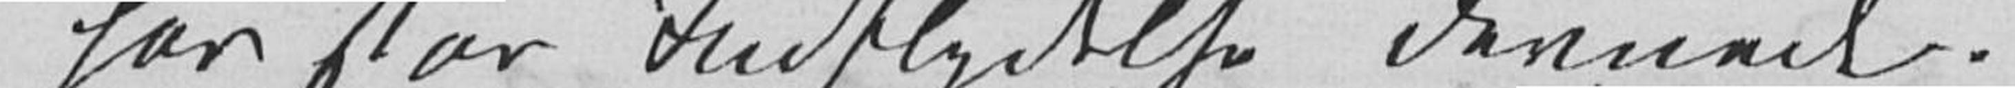har stor Indflydelse dernede.
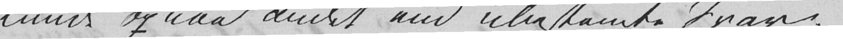kunde opnaa andet end ubestemte Svar.
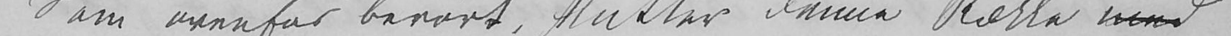Som ovenfor berørt, slutter denne Række med
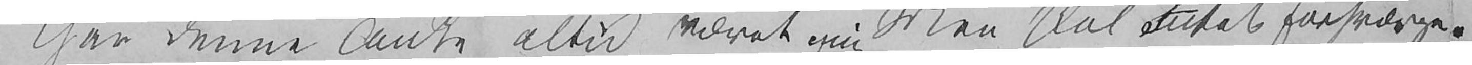har denne Tanke altid været mig. Man skal Intet forsværge.
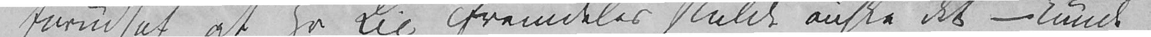forudsat at Hr Lie fremdeles skulde ønske det – kunde
